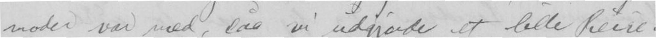moder var med, saa vi udgjorde et lille Reise-
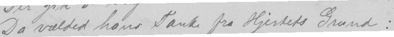Da vælded hans Tanke fra Hjertets Grund:
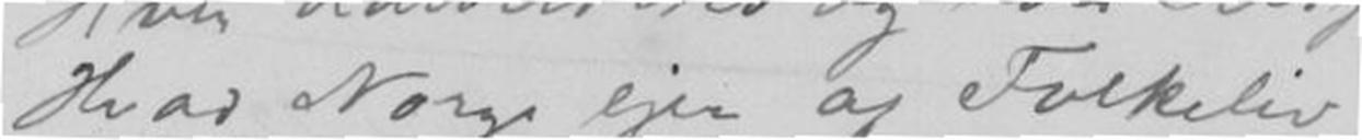Hvad Norge ejer af Folkeliv
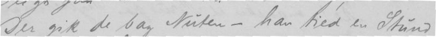Der gik de bag Nuten - han tied en Stund,
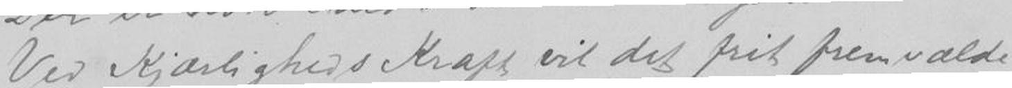Ved Kjærligheds Kraft vil det frit fremvælde,
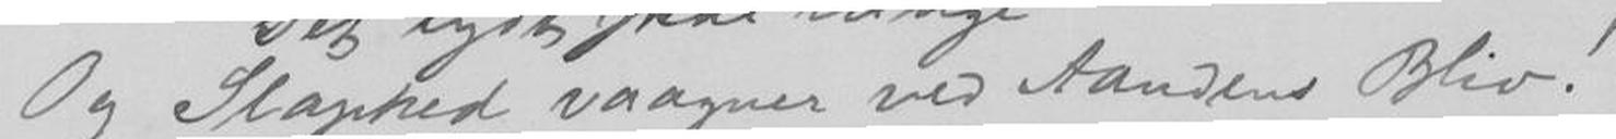Og Slaphed vaagner ved Aandens Bliv!
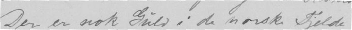Der er nok Guld i de norske Fjelde
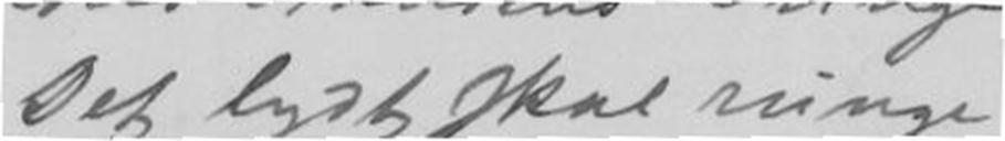Det lyst skal ringe
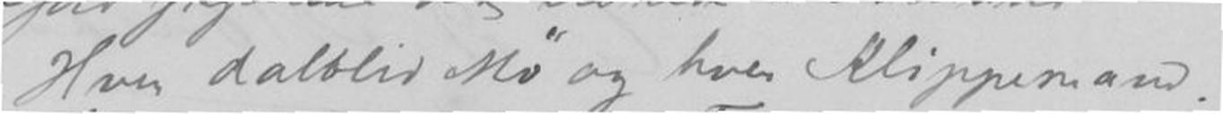Hver dalblid Mø og hver Klippemand.
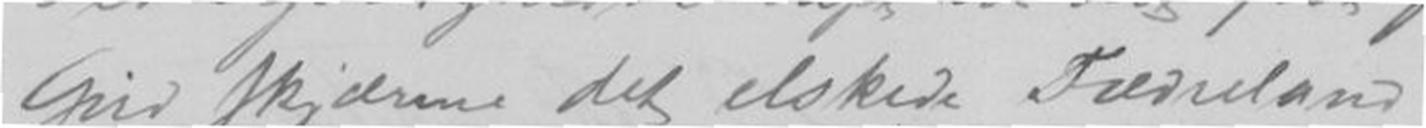Gud skjærmer det elskede Fædreland
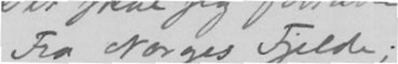Fra Norges Fjelde;
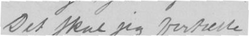Det skal jeg fortælle
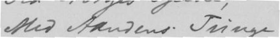Med Aandens runge
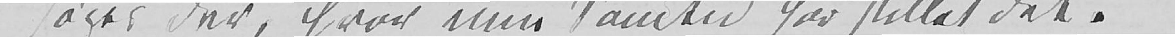søges der, hvor min Samtid har stillet det.
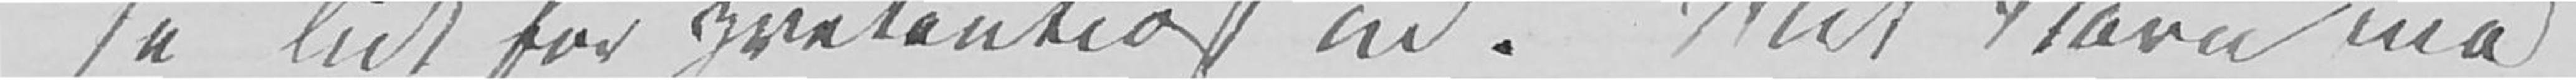se lidt for pretentiøst ud. Mit Navn må
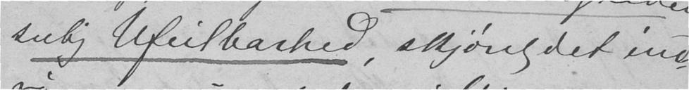subj Ufeilbarhed, skjønt det ind-
